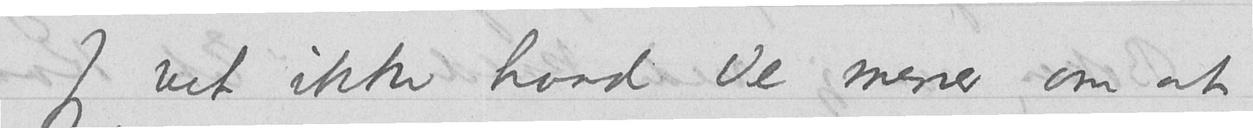Jeg vet ikke hvad De mener om at
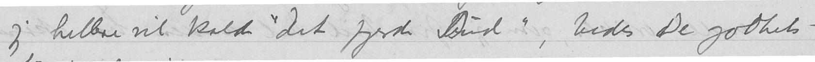jeg hellere vil kalde «Det fjerde Bud», bedes De godhets-
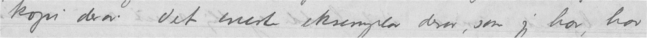kopi derav. Det eneste eksemplar derav, som jeg har, har
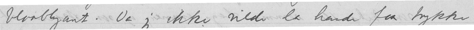blaablyant. Da jeg ikke vilde la hende faa trykke
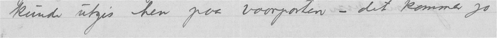kunde utgis hen paa vaarparten - det kommer jo
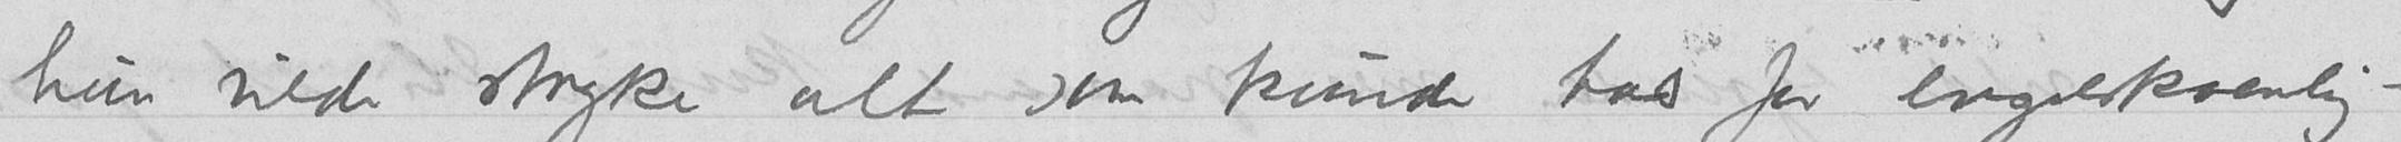hun vilde stryke alt som kunde tas for engelskvenlig-
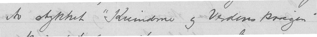Av stykket "Kvinderne og Verdenskrigen"
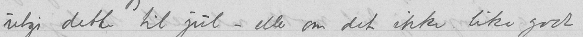utgi dette til jul - eller om det ikke like godt
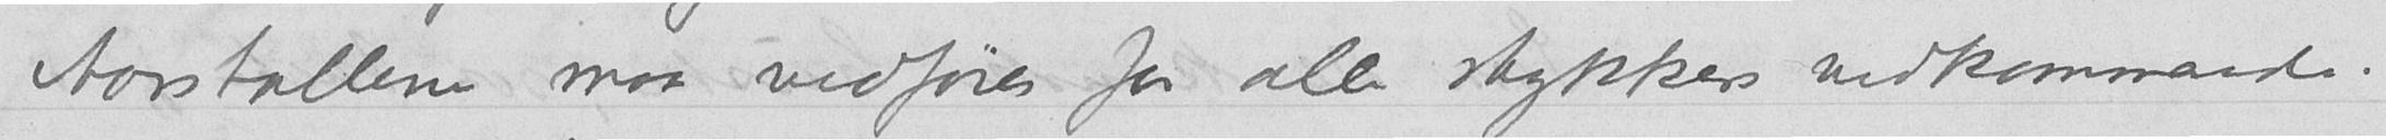Aarstallene maa vedføies for alle stykkers vedkommende.
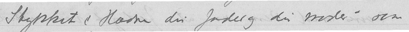Stykket "Hædre din fader og din moder" - som
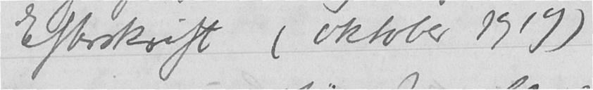Efterskrift (oktober 1919)
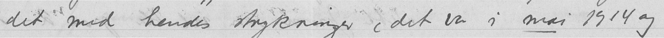det med hendes strykninger (det var i mai 1914 og
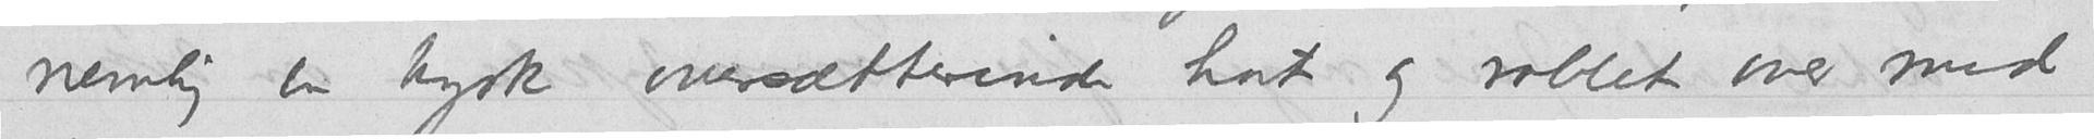nemlig en tysk oversætterinde hat og rablet over med
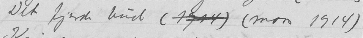Det fjerde bud (1904) (mars 1914)
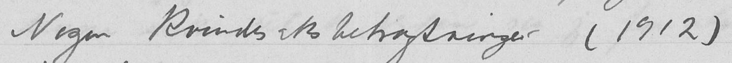Nogen Kvindesaksbetragtninger (1912)
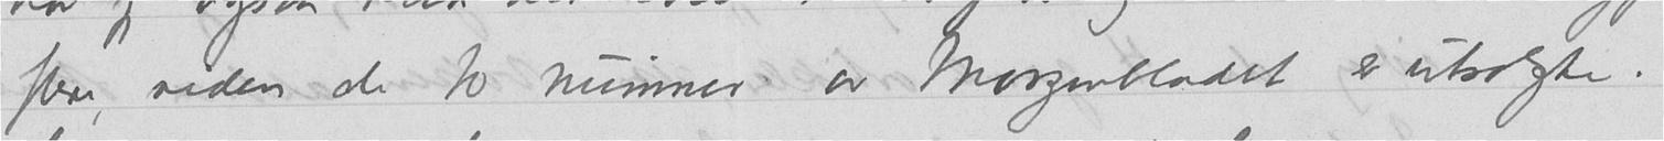flere, siden de to nummer av Morgenbladet er utsolgte.
